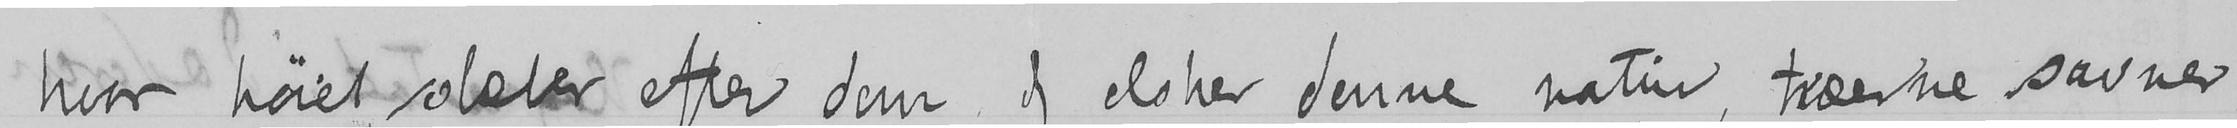hvor høiet slæber efter dem. Jeg elsker denne natur, træerne savner
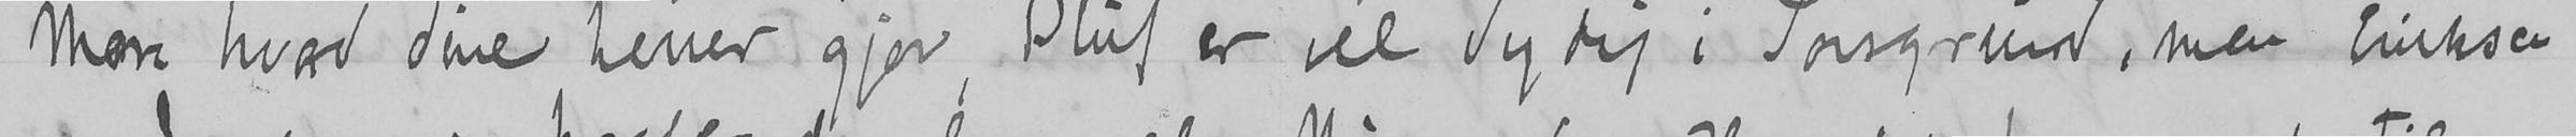Mon hvad dine herrer gjør, Oluf er vel dydig i Porsgrund, men Eriksen
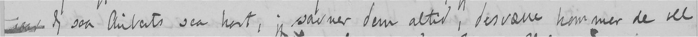iaar. Jeg saa Auberts saa kort, jeg savner dem altid, desvære kommer de vel
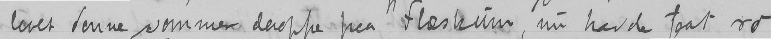levet denne sommer deroppe paa Flæskum, nu havde faat ro
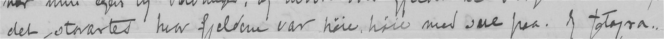det storartet hvor fjeldene var høie, høie med sne paa. Jeg fotogra-
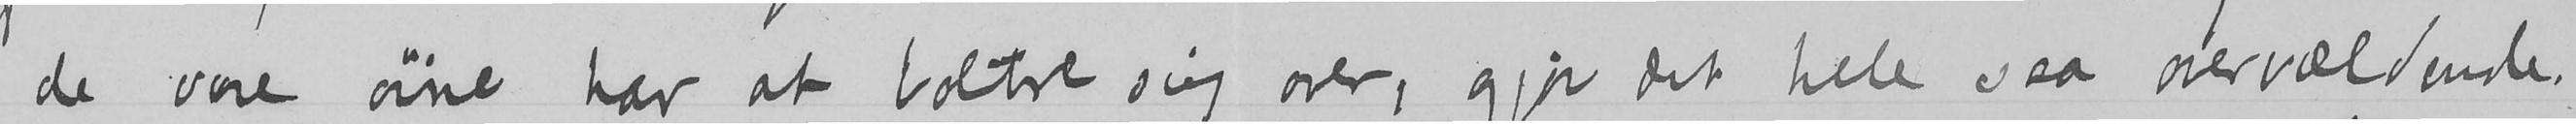de vore øine har at boltre sig over, gjør det hele saa overvældende.
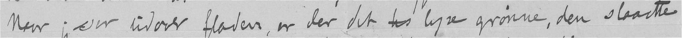Naar jeg ser udover fladen, er der det hs lyse grønne, den slaatte
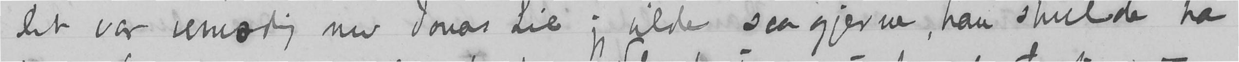Det var vemodig ned Jonas Lie jeg vilde saa gjerne, han skulde ha
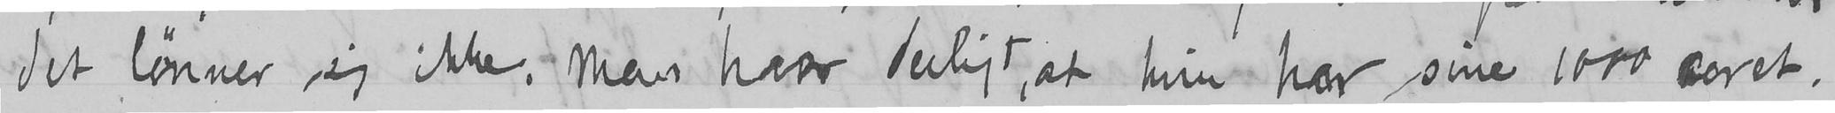det lønner sig ikke. Men hvor deiligt, at hun har sine 1000 aaret.
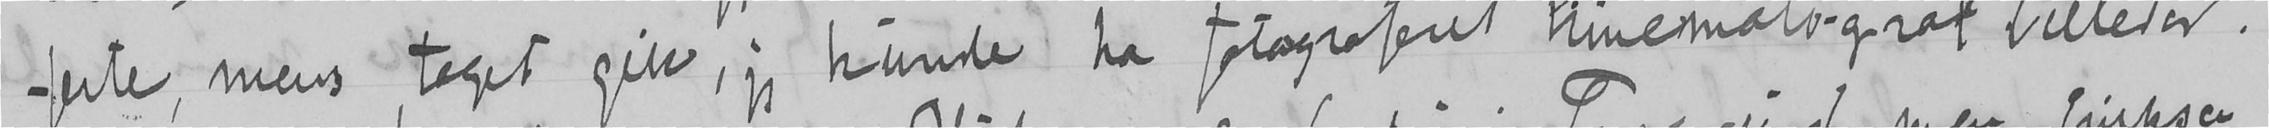ferte, mens toget gik, jeg kunde ha fotograferet kinematografbilleder.
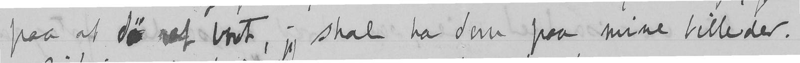paa at dø af brot, jeg skal ha dem paa mine billeder.
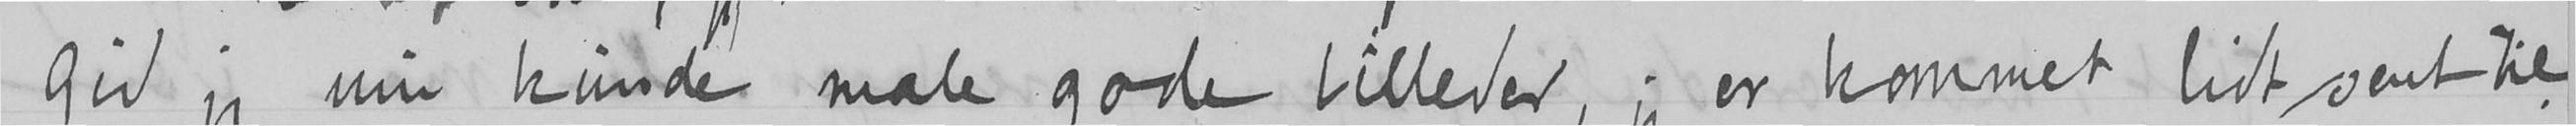Gid jeg nu kunde male gode billeder, jeg er kommet lidt sent til
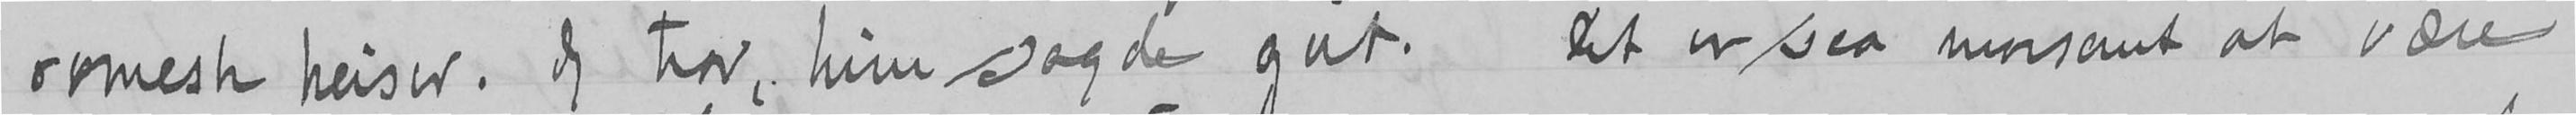romersk keiser. Jeg tror, hun sagde gut. Det er saa morsomt at være
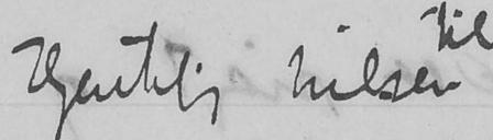Hjertelig hilsen
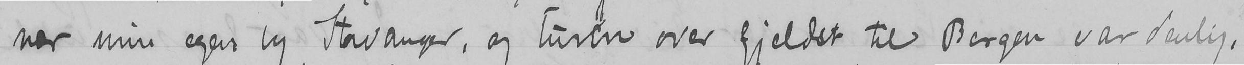nær min egen by Stavanger, og turten over fjeldet til Bergen var deilig,
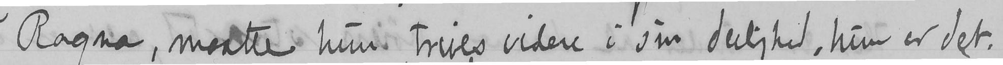Ragna, maatte hun trives videre i sin deilighed, hun er det.
